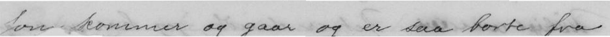som kommer og gaar og er saa borte fra
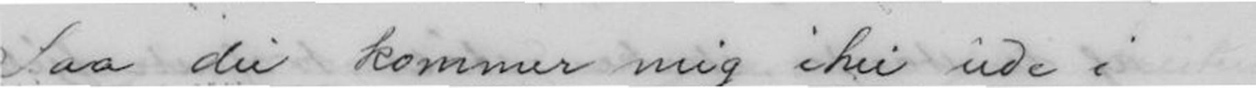Saa du kommer mig ihu ude i
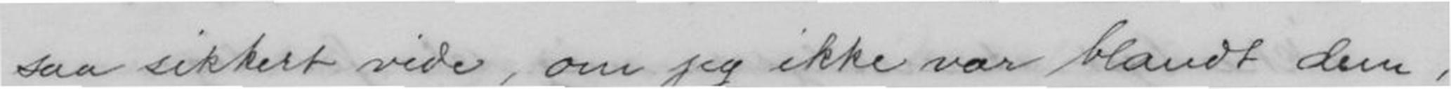saa sikkert vide, om jeg ikke var blandt dem,
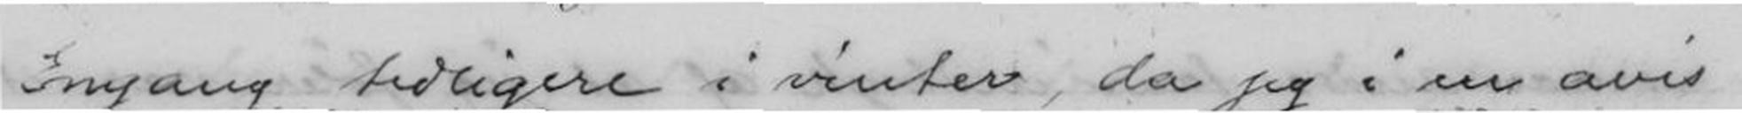Engang tidligere i vinter, da jeg i en avis
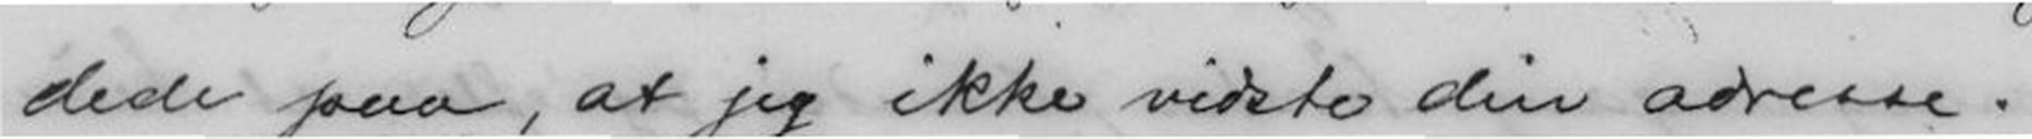dede paa, at jeg ikke vidste din adresse.
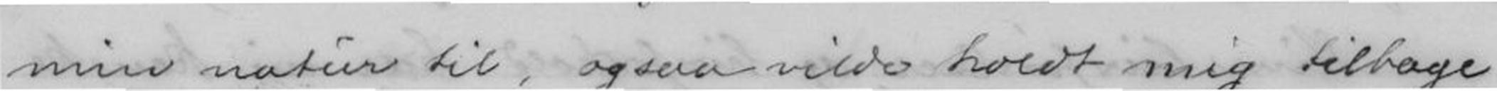min natur til, ogsaa vilde holdt mig tilbage
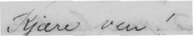Kjære ven!
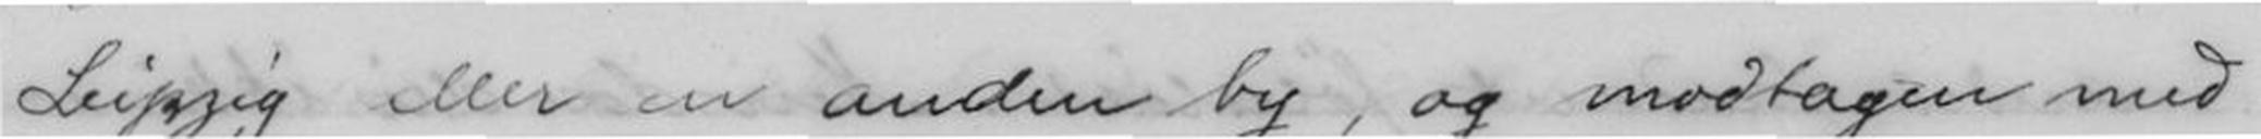Leipzig eller en anden by, og modtagen med
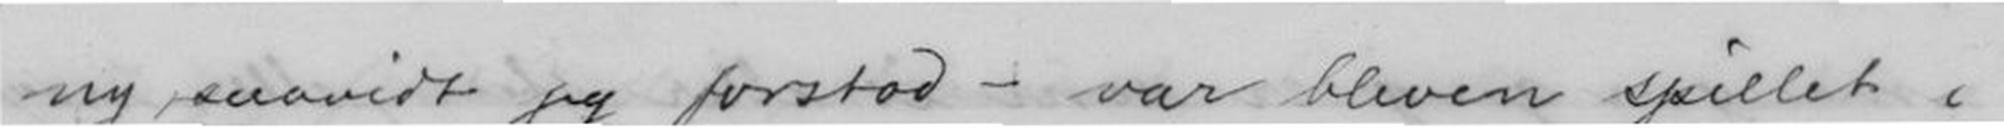ny saavidt jeg forstod - var bleven spillet i
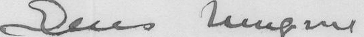Deres Hengivne
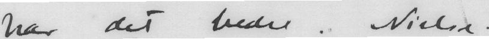har det bedre. Nielse
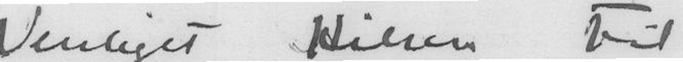Venligst Hilsen til
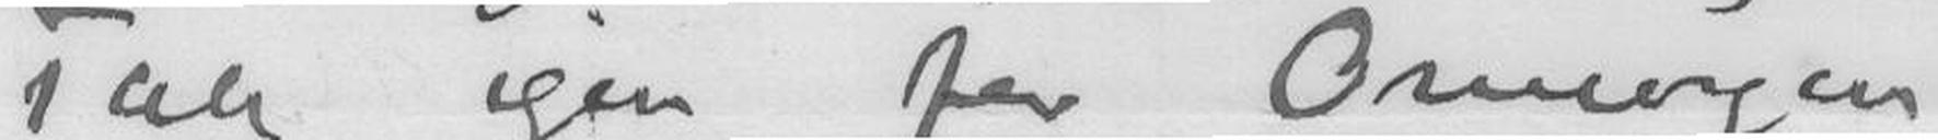Tak igen for Omsorgen
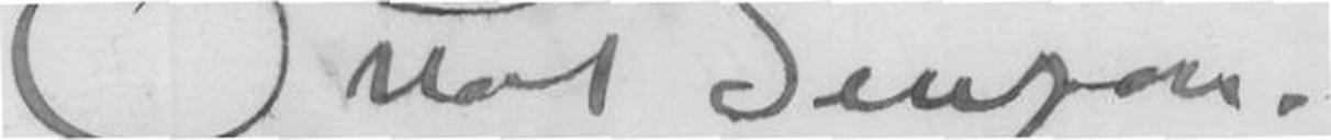Otto Benzon.
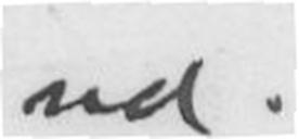ud.
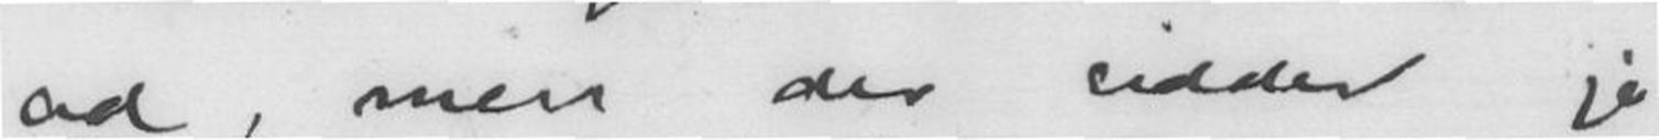ad, men der sidder jo
